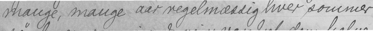mange, mange aar regelmæssig hver sommer
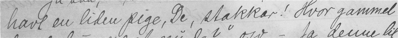havt en liden pige, De, stakkar! Hvor gammel
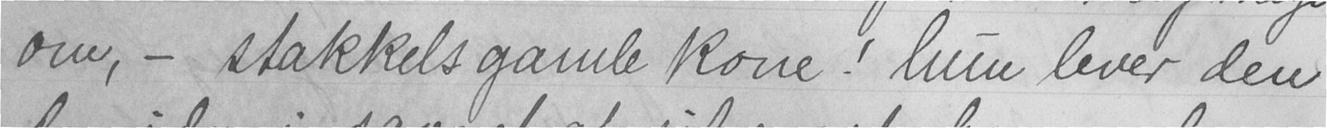om, - stakkels gamle kone! hun lever den
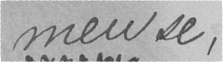men se,
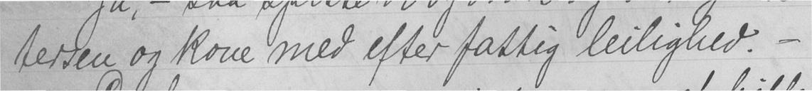tersen og kone med efter fattig leilighed. -
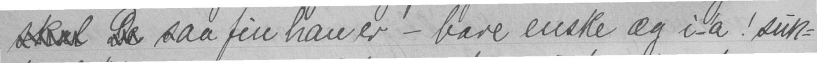skal De saa fin han er - bare enske æg i-a! suk-
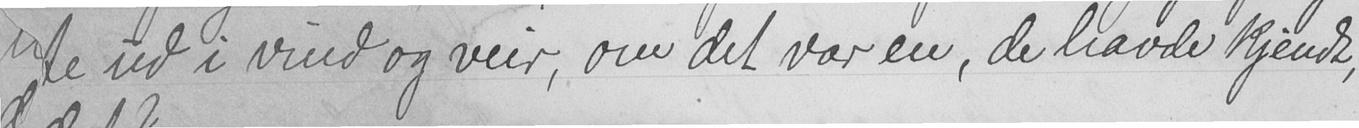te ud i vind og veir, om det var en, de havde kjendt,
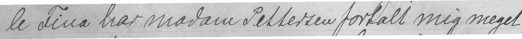le Tina har madam Pettersen fortalt mig meget
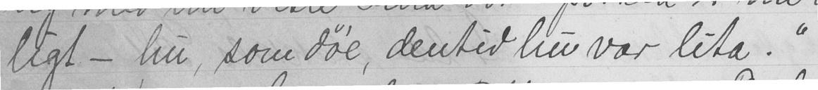ligt - hu, som døe, dentid hu var lita. "
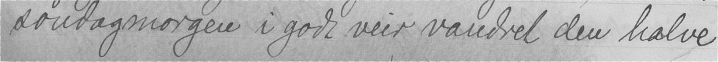søndagmorgen i godt veir vandret den halve
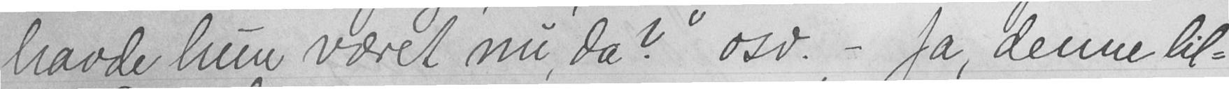havde hun været nu, da?" osv. - Ja, denne lil-
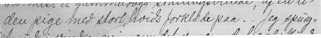den pige med stort, hvidt forklæde paa. - Jeg spurg-
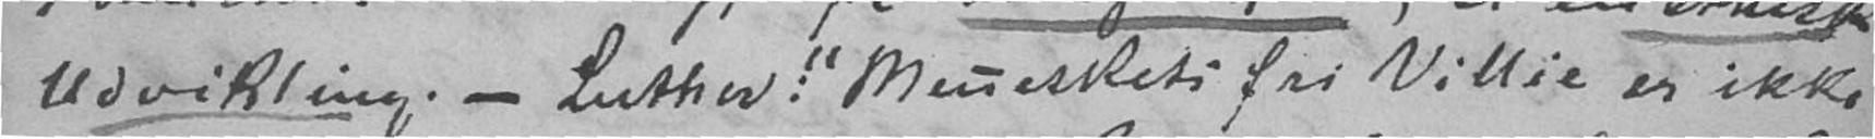Udvikling. - Luther: "Menneskets fri Villie er ikke
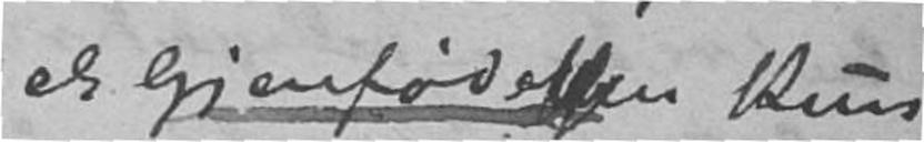el Gjenfødelsen kun
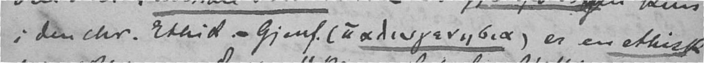i den chr. Ethik – Gjenf (gresk) er en ethisk
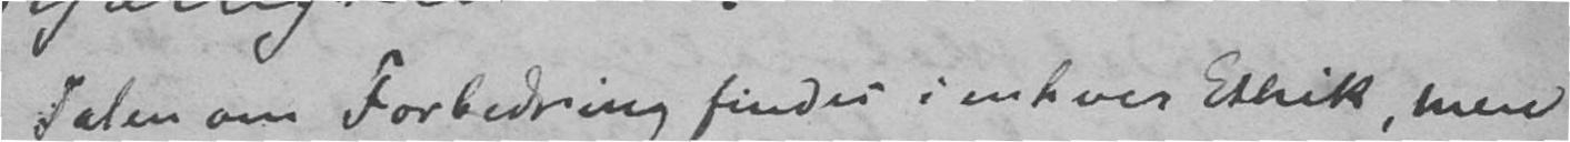Talen om Forbedring findes i enhver Ethik, men
